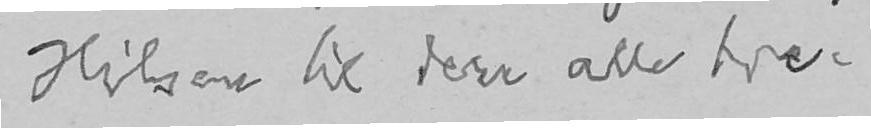Hilsen til dere alle tre.
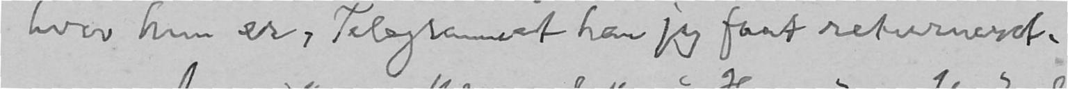hvor hun er, Telegrammet har jeg faat returneret.
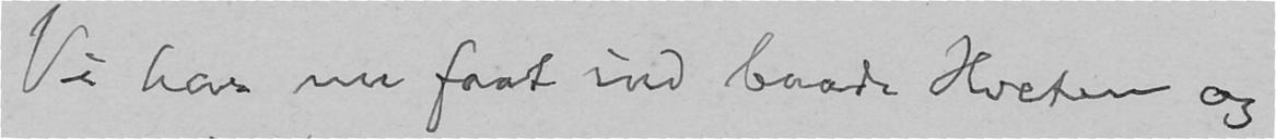Vi har nu faat ind baade Hveten og
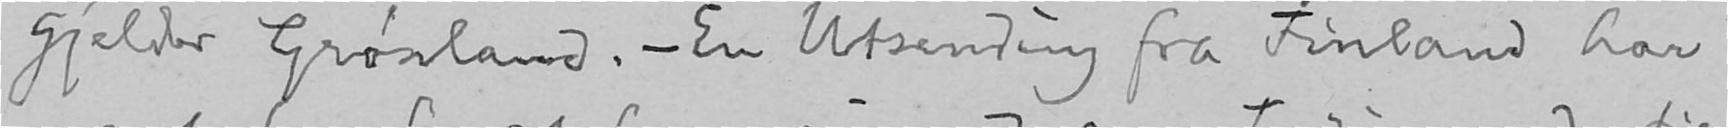gjelder Grønland. - En Utsending fra Finland har
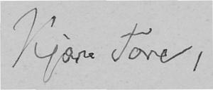Kjære Tore,
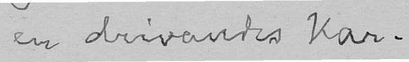en drivandes Kar.
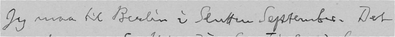Jeg maa til Berlin i Slutten September. Det
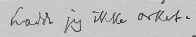hadde jeg ikke orket.
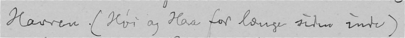Havren .(Høi og Haa for længe siden inde)
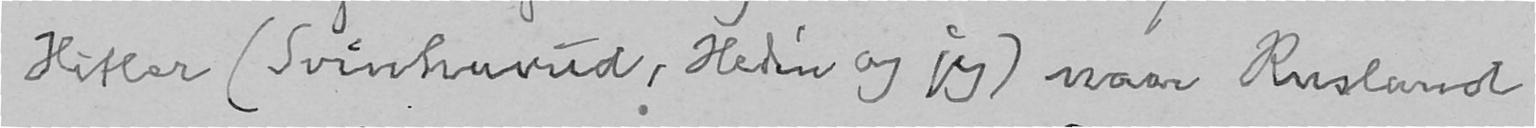Hitler (Svinhuvud, Hedin og jeg) naar Rusland
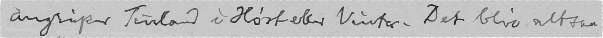angriper Finland i Høst eller Vinter. Det blir altsaa
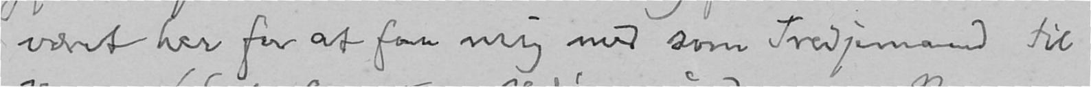været her for at faa mig med som Tredjemand til
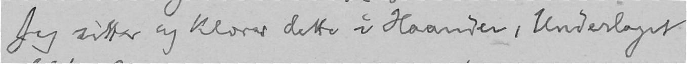Jeg sitter og klorer dette i Haanden, Underlaget
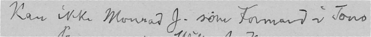Kan ikke Monrad J. som Formand i Tono
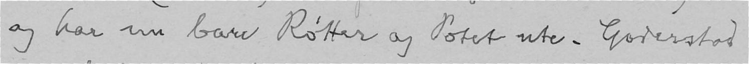og har nu bare Røtter og Potet ute. Goderstad
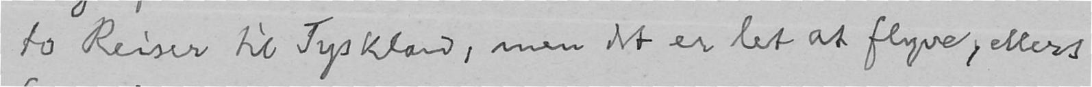to Reiser til Tyskland, men det er let at flyve, ellers
